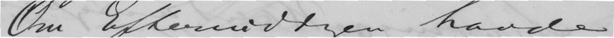Om Eftermiddagen havde
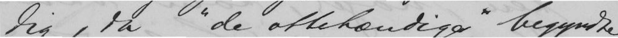dig, da "de ottehændige" begyndte
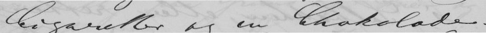Cigaretter og en Chokolade-
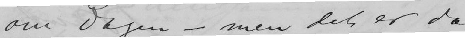om Dagen - men det er dog
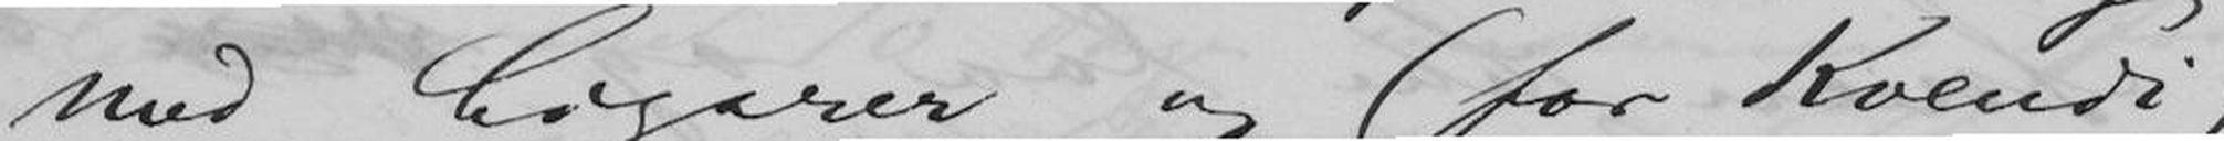med Cigarer og (for Kvendi)
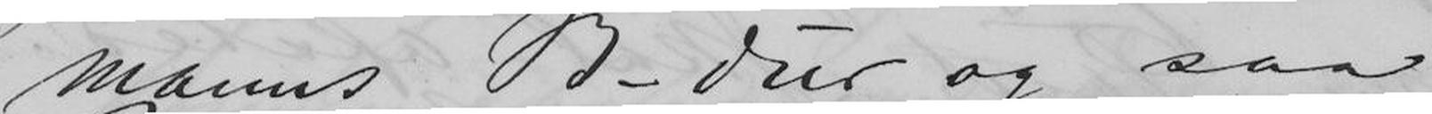manns B-dur og saa
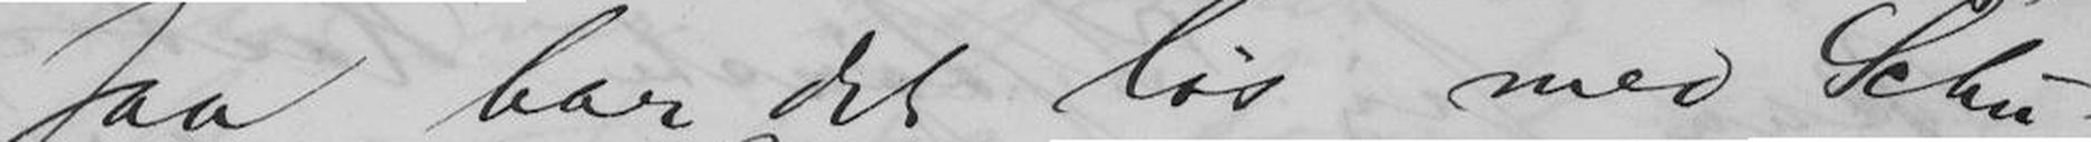saa bar det løs med Schu-
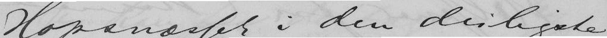Hopsnæsset i den deiligste
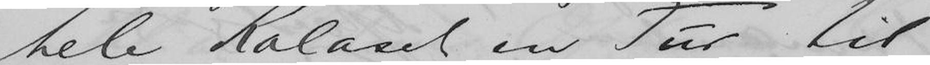hele Kalaset en Tur til
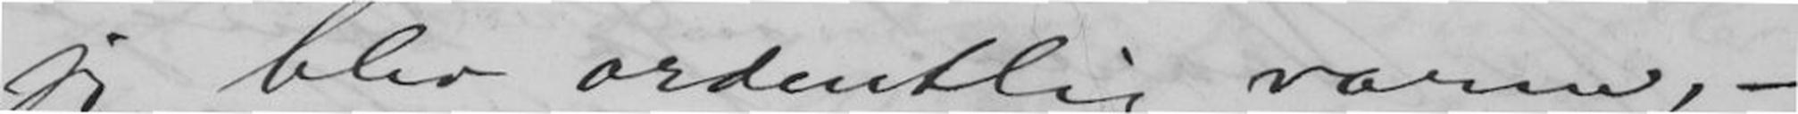jeg blev ordentlig varm, -
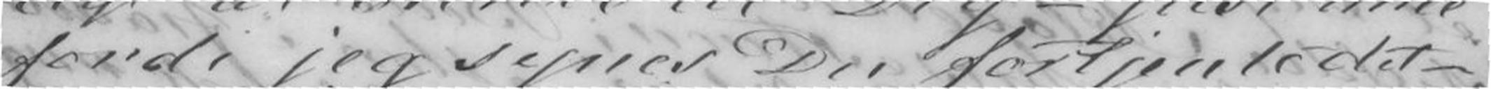fordi jeg synes Du fortjente det -
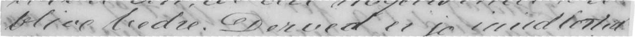blive bedre. Derved er jo imidlertid
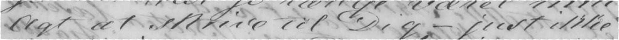Agt at skrive til Dig - just ikke
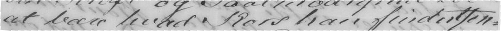at bære hvad Kors han finder tjen-
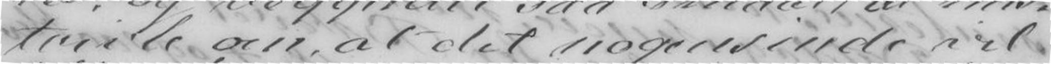tvivle om, at det nogensinde vil
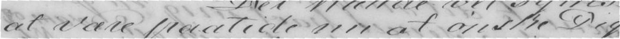at være paatide nu at ønske Dig
